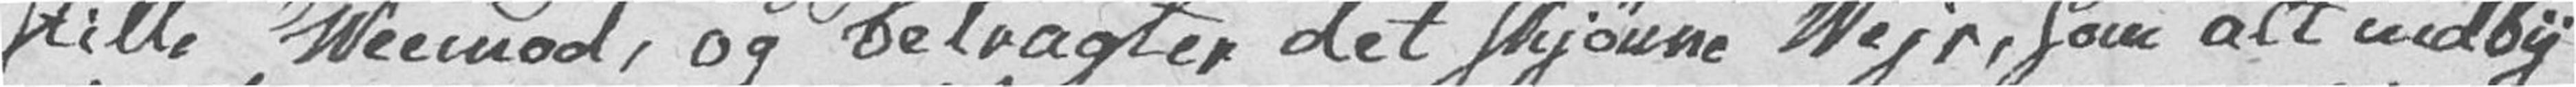stille Veemod, og betragter det skjønne Vejr, som alt indby
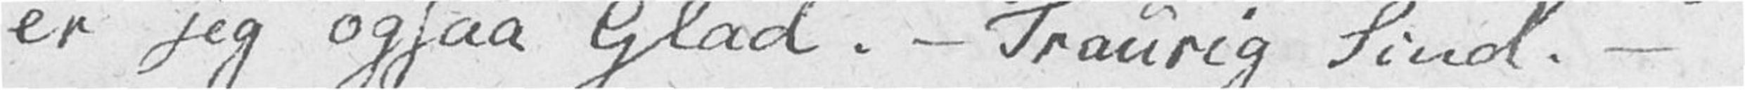er jeg ogsaa Glad. – Traurig Sind. –
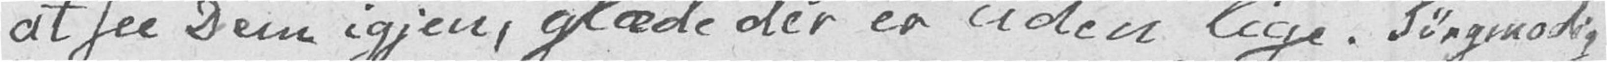at see Dem igjen, glæde der er uden lige. Sørgmodig.
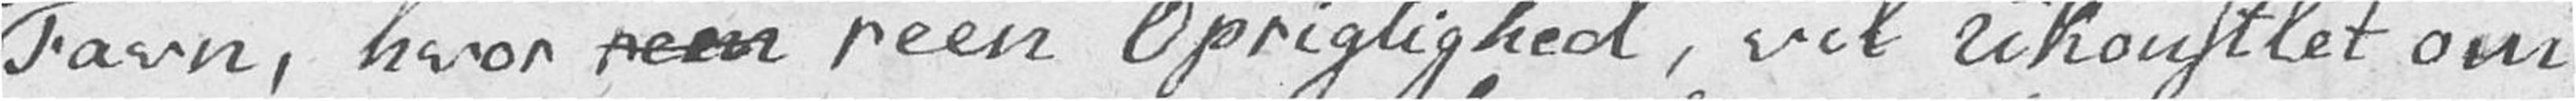Favn, hvor reen reen Oprigtighed, vil Ukonstlet om
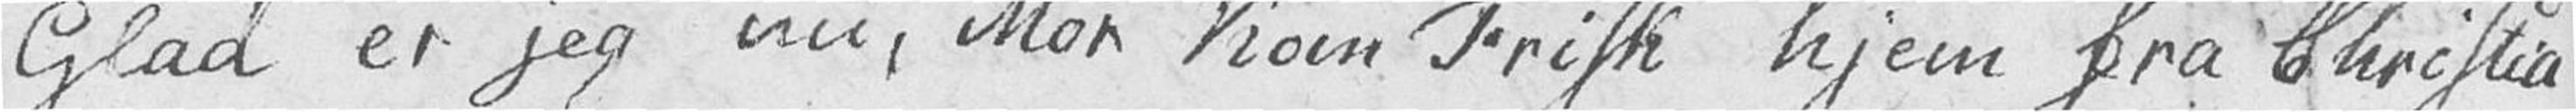Glad er jeg nu, Mor kom Frisk hjem fra Christia
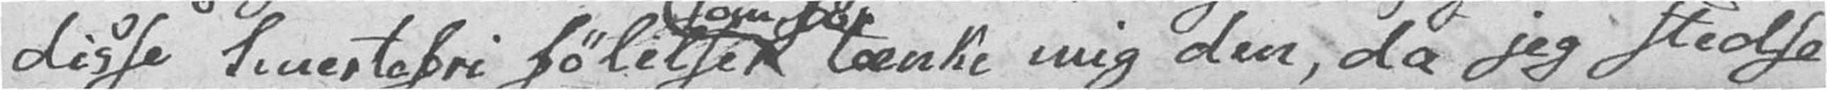disse Smertefri følelser som før tænke mig den, da jeg stedse
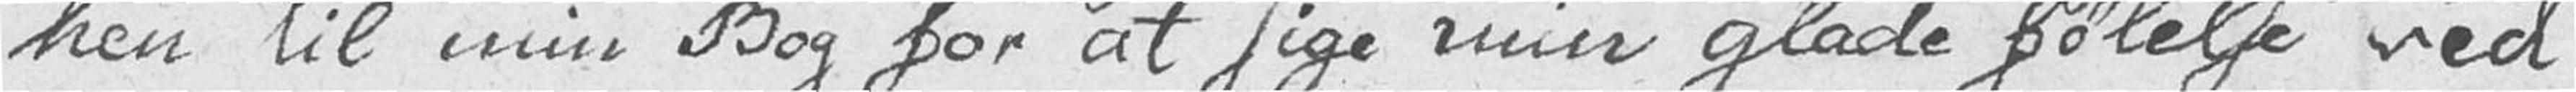hen til min Bog for at sige min glade følelse ved
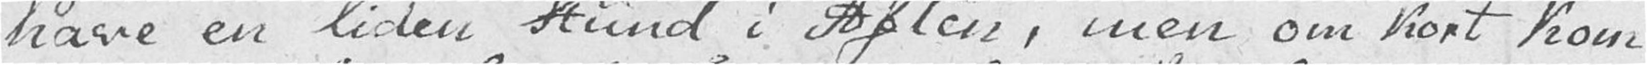have en liden Stund i Aften, men om kort kom
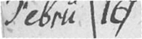Febru 17
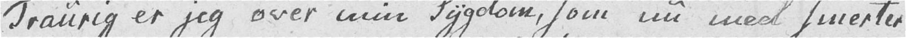Traurig er jeg over min Sygdom, som nu med smerter
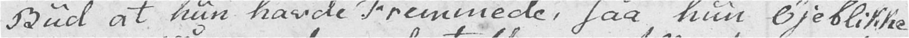Bud at hun havde Fremmede, saa hun Øjeblikke
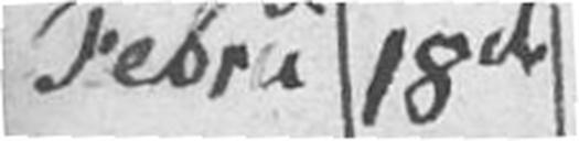Febru 18de
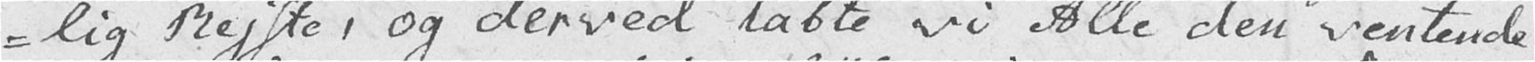-lig Rejste, og derved tabte vi Alle den ventende
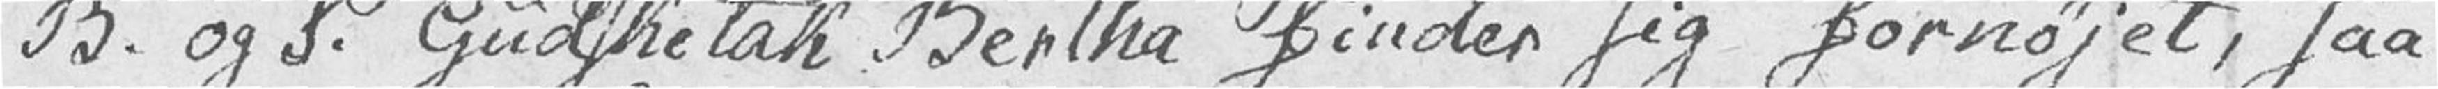B. og S. Gudsketak Bertha finder sig fornøjet, saa
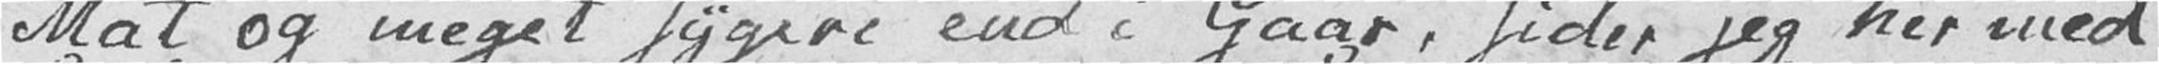Mat og meget sygere en i Gaar, sider jeg her med
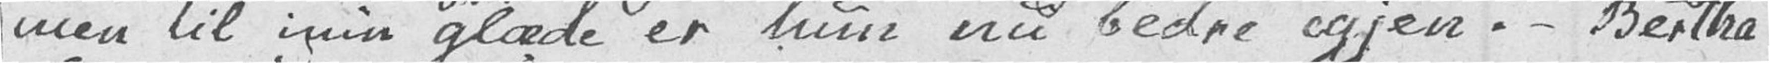men til min glæde er hun nu bedre igjen. – Bertha
